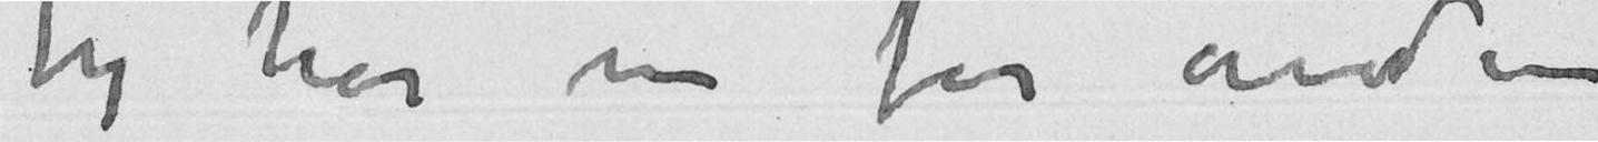Jeg har nu for anden
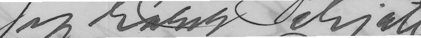Jeg hører Schjøtt
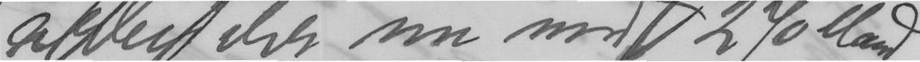arbeider nu med 270 Mand
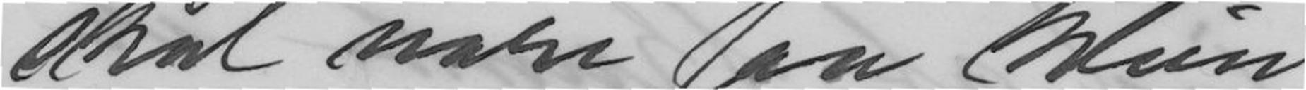skal være saa klein.
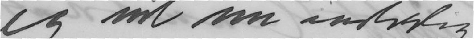Jeg vil nu inderlig
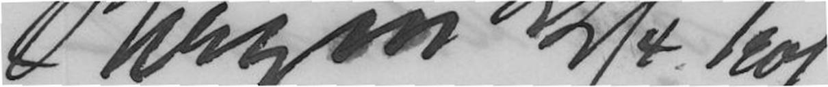Bergen 22/4 1901
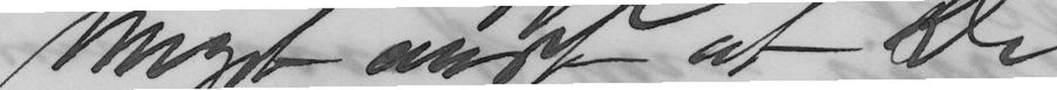meget ondt at De
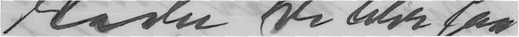haabe De blir saa
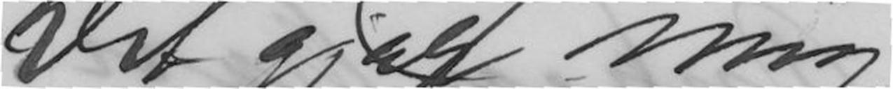Det gjør mig
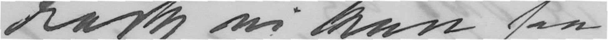rask vi kan faa
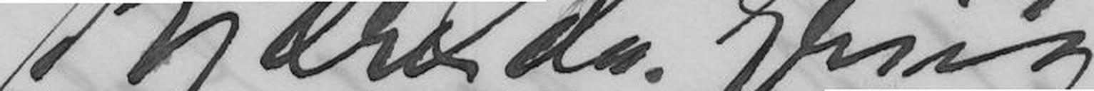Kjære Edv. Grieg
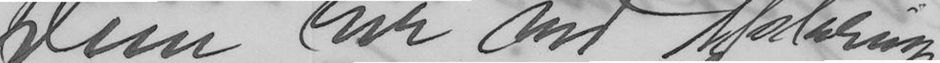Dem her ved Afsløringen.
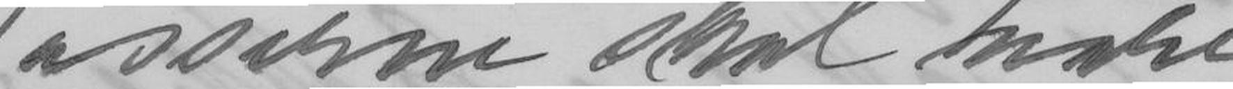Basserne skal være
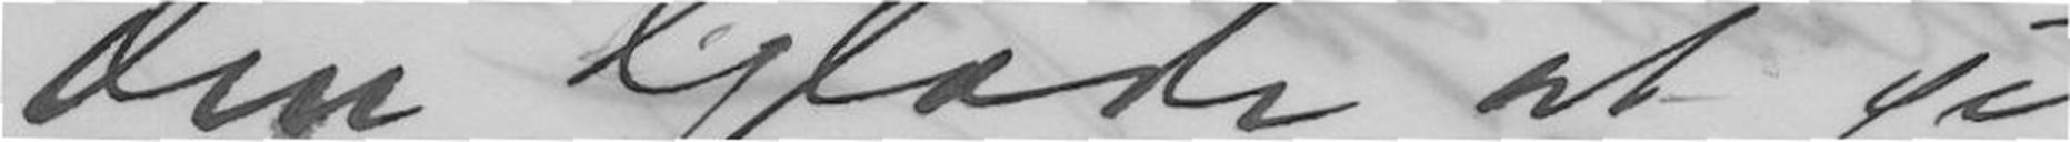den Glæde at se
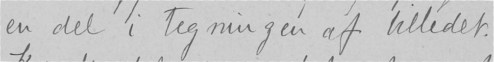en del i tegningen af billedet.
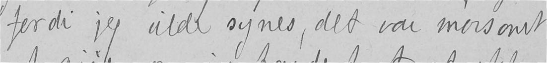fordi jeg vilde synes, det var morsomt
